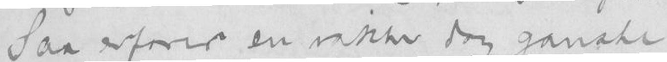Saa erfarer en vakker dag ganske
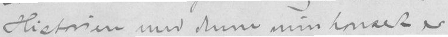Historien med denne min konsert er
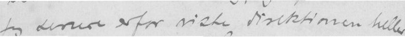jeg senere erfor viste direktionen heller
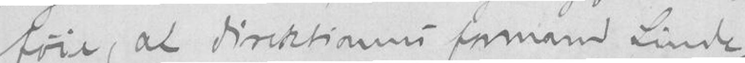føie, at direktionens formand Linde
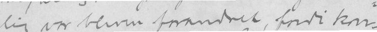lig var bleven forandret, fordi kon-
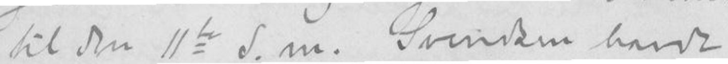til den 11te d.m. Svendsen havde
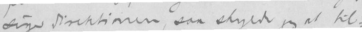siger direktionen, saa skylder jeg at til-
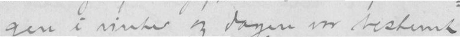gen i vinter og dagen var bestemt
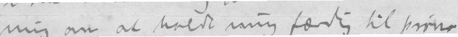mig om at holde mig færdig til prøver.
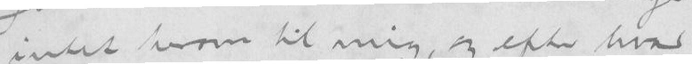intet herom til mig, og efter hvad
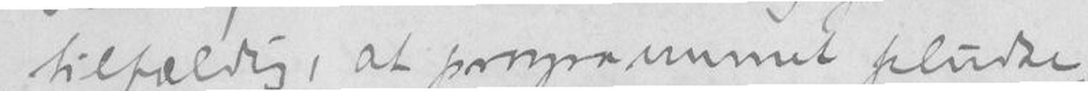tilfældig, at programmet pludse-
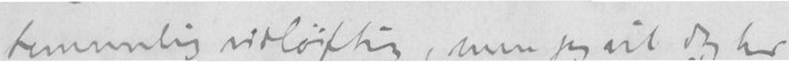temmelig vidløftig, men jeg vil dog her
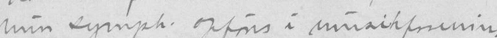min symph. opføres i musikforenin-
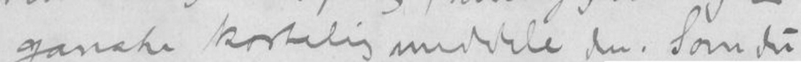ganske kortelig meddele den. Som du
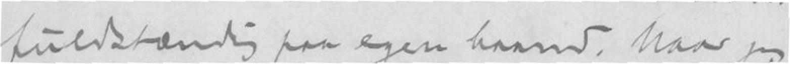fulstændig paa egen haand. Naar jeg
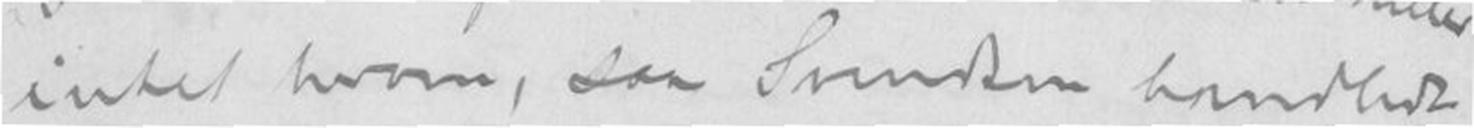intet herom, saa Svendsen handlede
